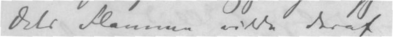dets Flamme vilde deraf
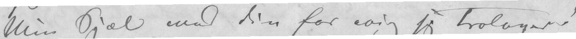Min Sjæl med din for evig jeg trolover!
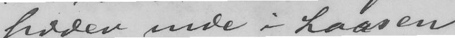sidder inde i Laasen.
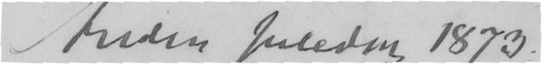Anden Juledag 1873
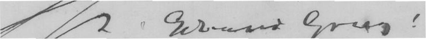Hr Edvard Grieg!
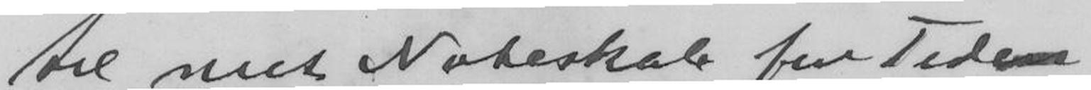til mit Noteskab for Tien
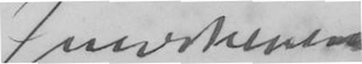Joh D Behrens
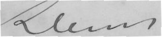Klem
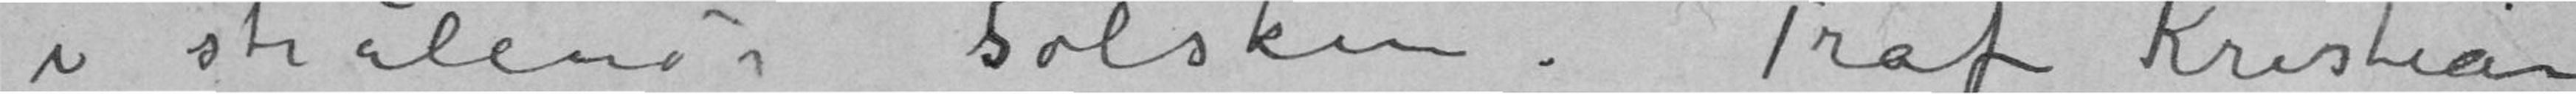i strålende Solskin. Traf Kristian
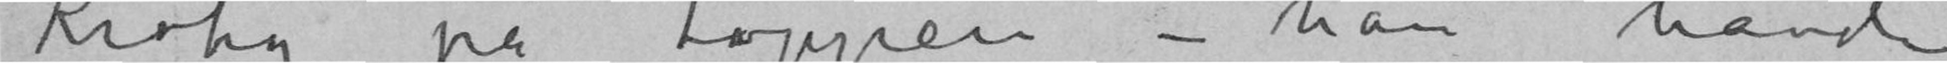Krohg på Toppen – han havde
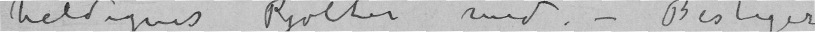heldigvis Pjolter med. – Bestiger
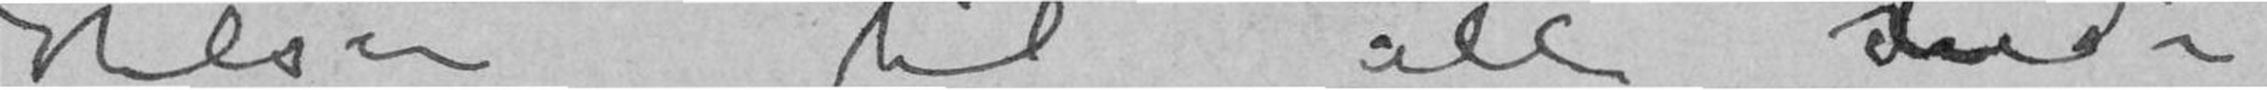Hilsen til alle nede
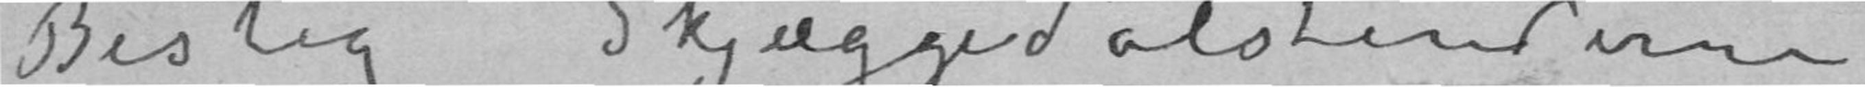Besteg Skjæggedalstinderne
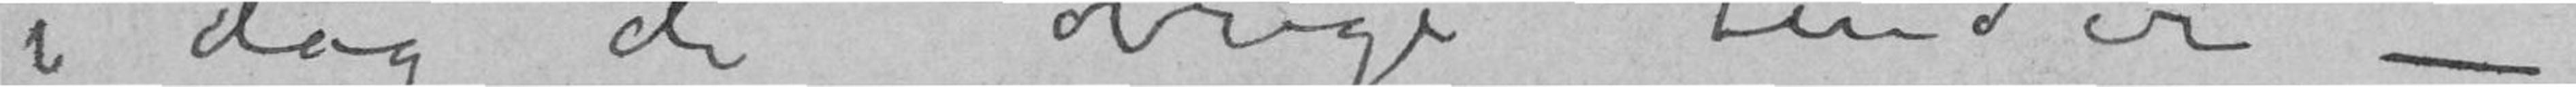i dag de øvrige Tinder –
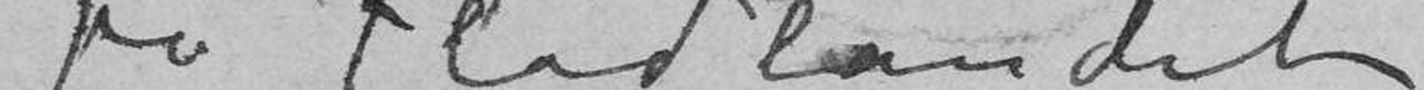på Fladlandet
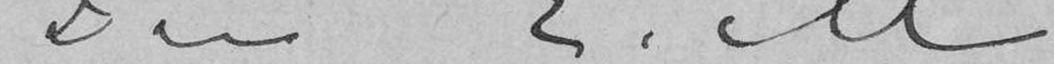Din E. M
